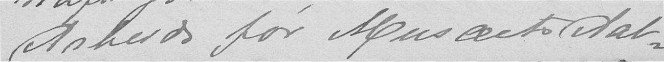Arbeide før Musæets Aab-
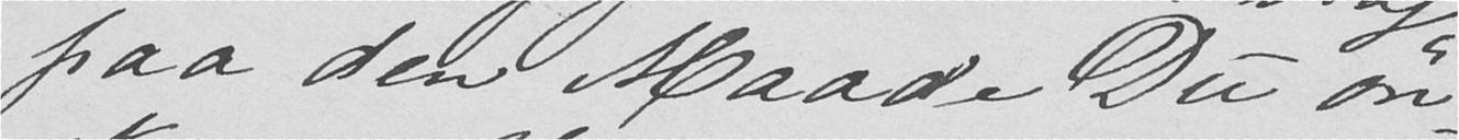paa den Maade Du øn-
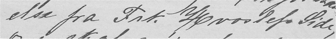else fra Frk. Hvoslefs Side,
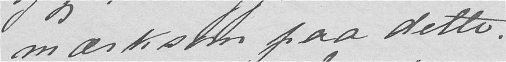mærksom paa dette.
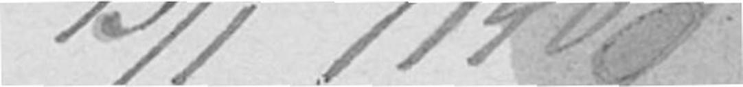13/1 1908
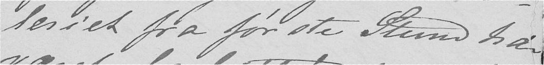leriet fra første Stund har
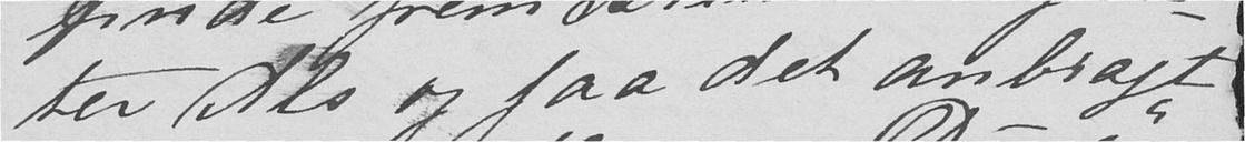ter Als og faa det anbragt
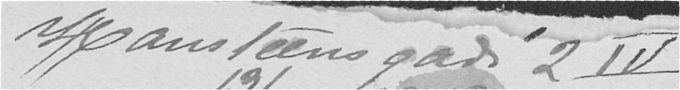Hansteens gade 2 IV
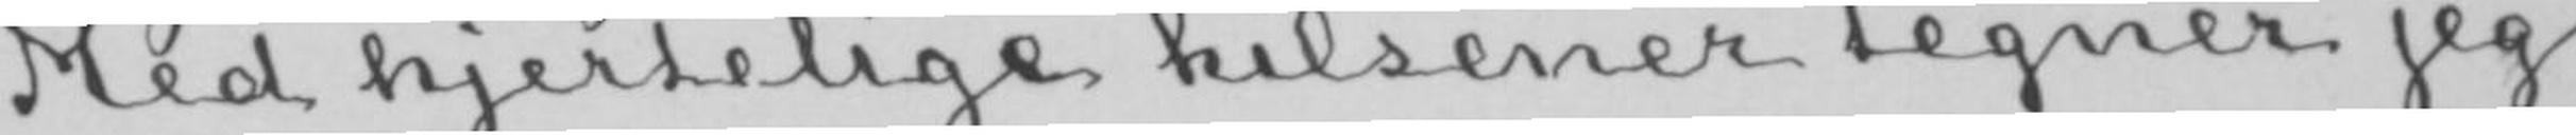Med hjertelige hilsener tegner jeg
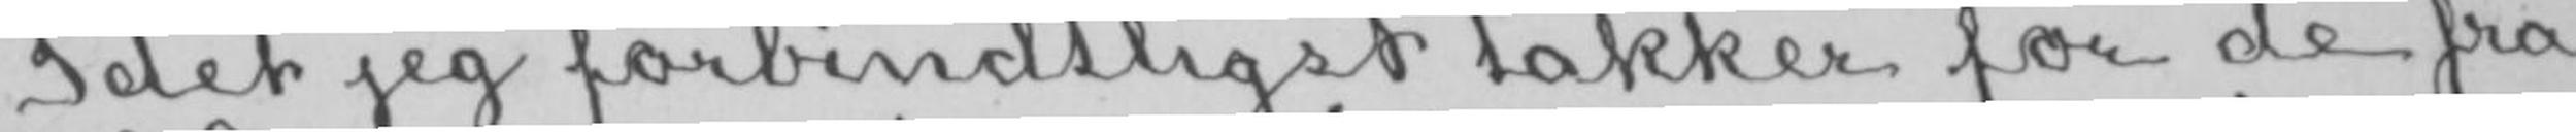Idet jeg forbindtligst takker for de fra
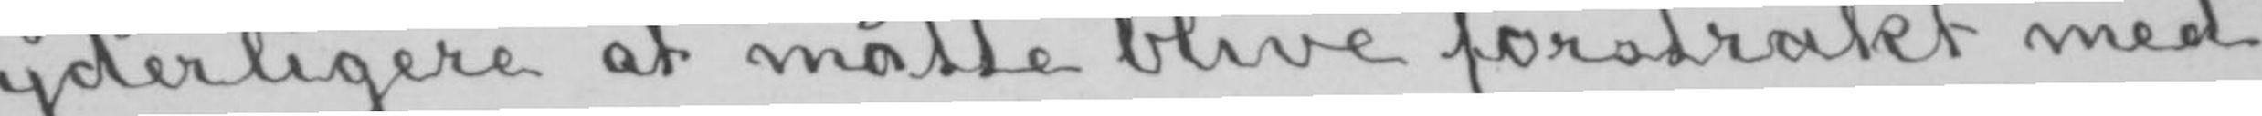yderligere at måtte blive forstrakt med
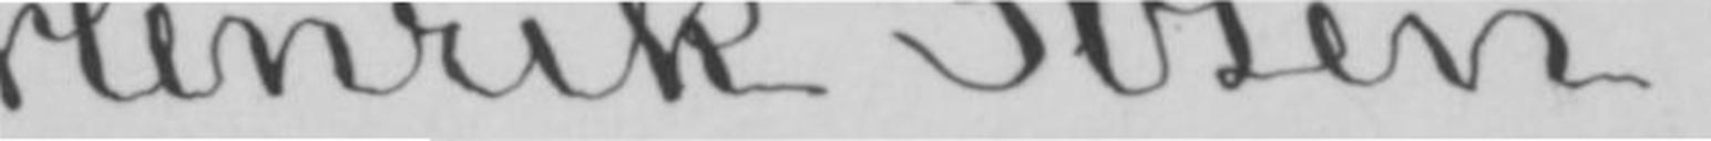Henrik Ibsen.
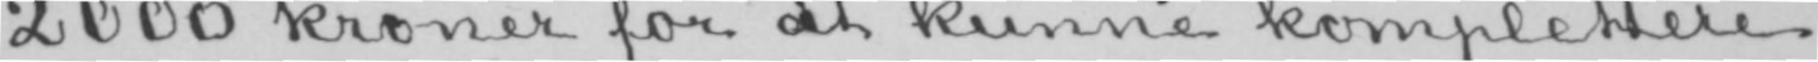2000 kroner for dat kunne komplettere
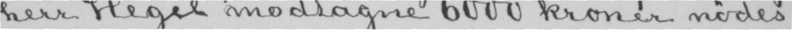herr Hegel modtagne 6000 kroner nødes
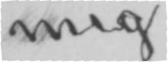mig
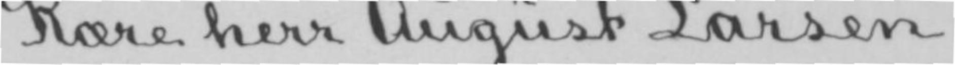Kære herr August Larsen,
